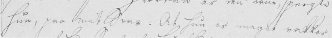hun, paa mit Svar: At hun er meget vakker
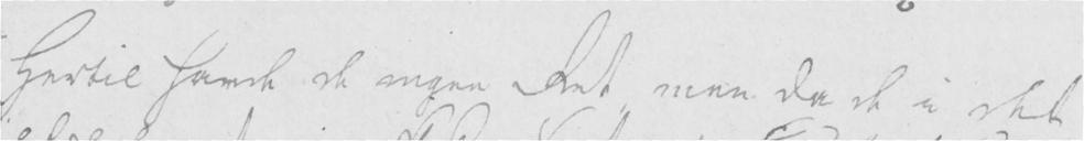Hertil havde de ingen Ret men da de i det
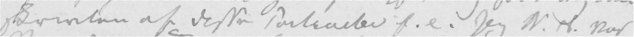skrivten af disse Portraiter f. e. seg N. N. var
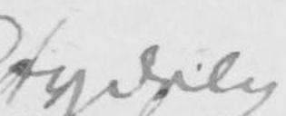tydelig
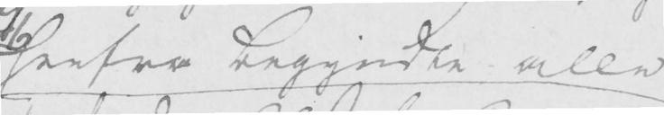herfra begyndte alle
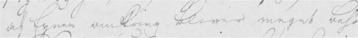at Egnen omkring bliver meget be
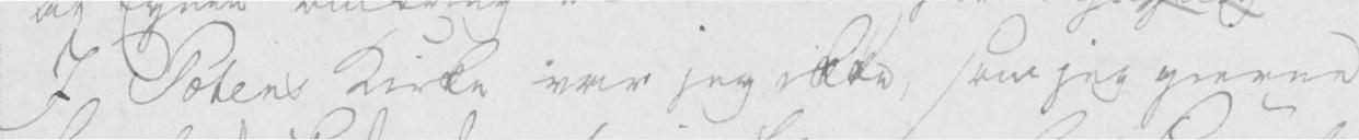I Totens Kirke var jeg ikke, som jeg gierne
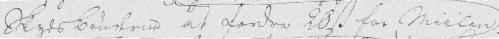Skyds Bønderne at fordre 20 s for Miilen
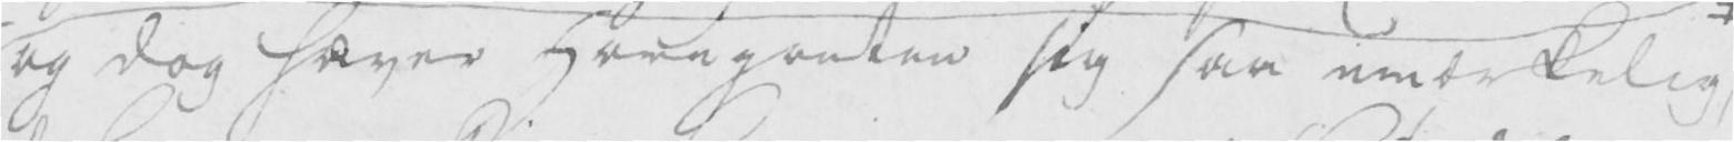og dog hæver Hovegaaten sig saa umærkelig
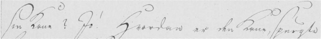sin Kone? Jo! Hvordan er den Kone, spurgte
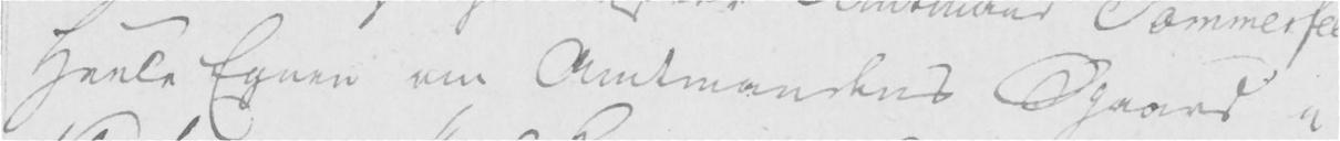Heele Egnen om Amtmandens Gaard i
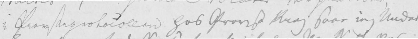i Provsteprotocollen hos Provst Krog faar ing Under-
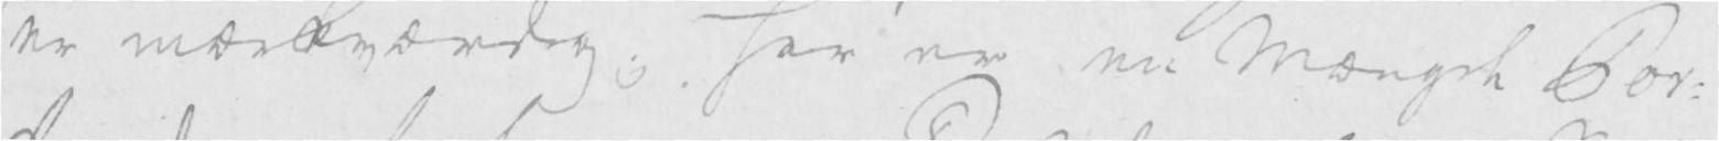er mærkværdig; her er en mængde Por-
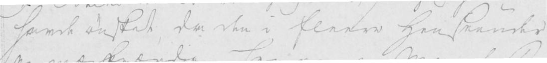havde ønsket da den i fleere Henseende
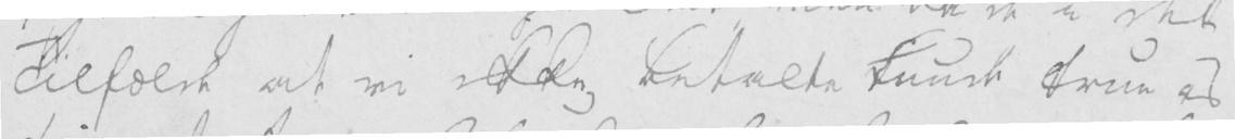tilfælde at vi ikke betalte kunde true os
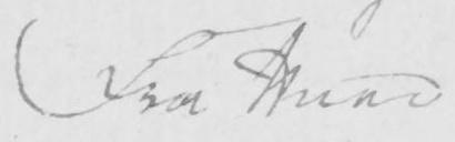Fra Wund
